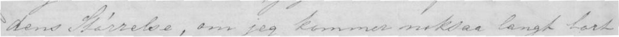dens Størelse, om jeg kommer noksaa langt bort
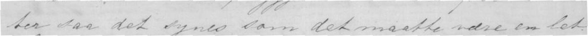ter saa det synes som det maatte være en let
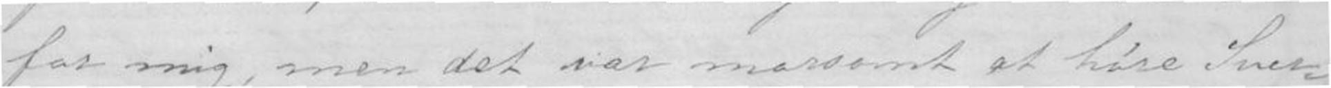for mig, men det var morsomt at høre Sver-
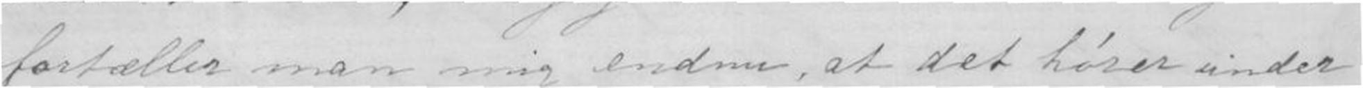fortæller men mig endnu, at det hører under
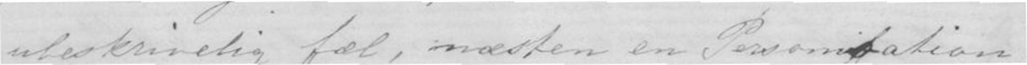ubeskrivelig fæl, næsten en Personifation
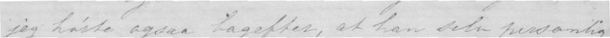jeg hørte ogsaa bagefter, at han selv personlig
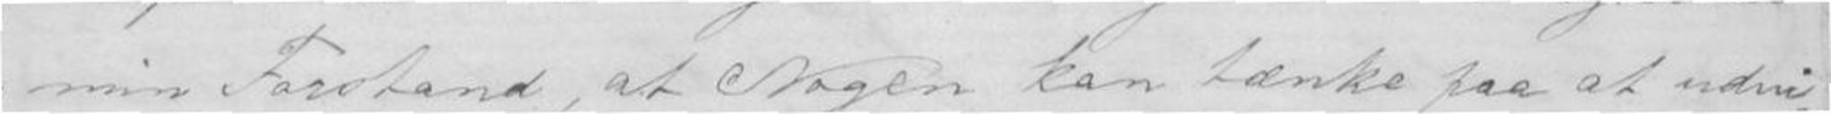min Forstand, at Nogen kan tænke paa at udvi-
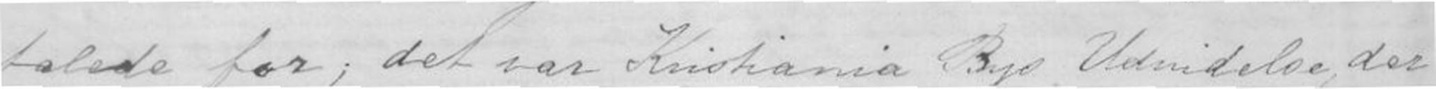talede for; det vat Kristiania Bys Udvidelse der
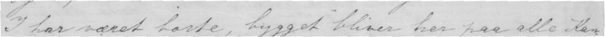I har været borte, bygget bliver har paa alle Kan-
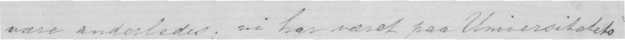være anderledes; vi har været paa Universitetets
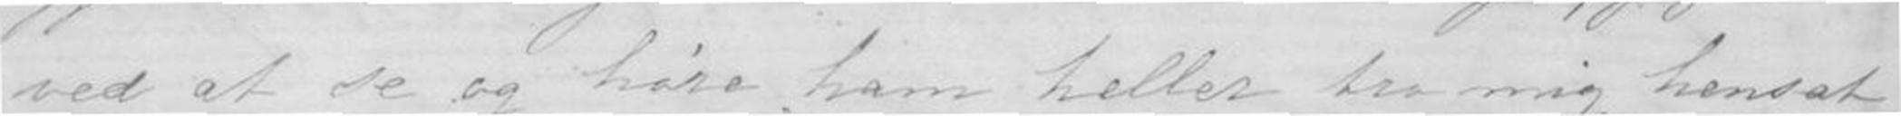ved at se og høre ham heller tro mig henset
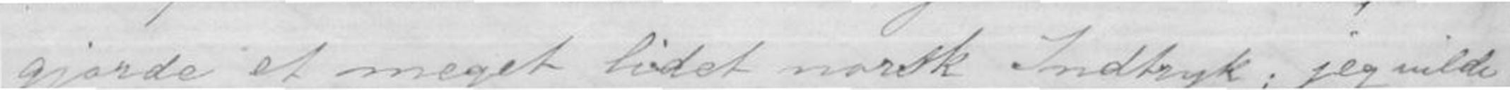gjorde et meget lidet norsk Intryk; jeg vilde
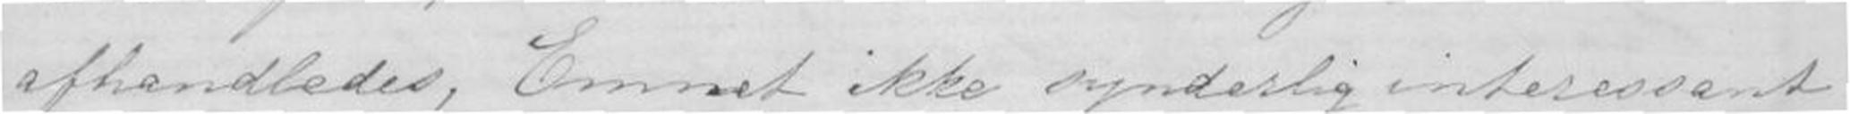afhandledes, Emnet ikke synderlig interessant
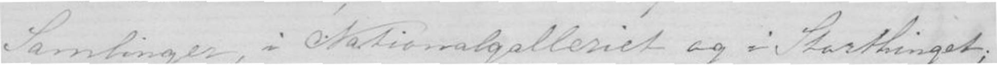Samlinger, i Nationalgalleriet og i Storthinget;
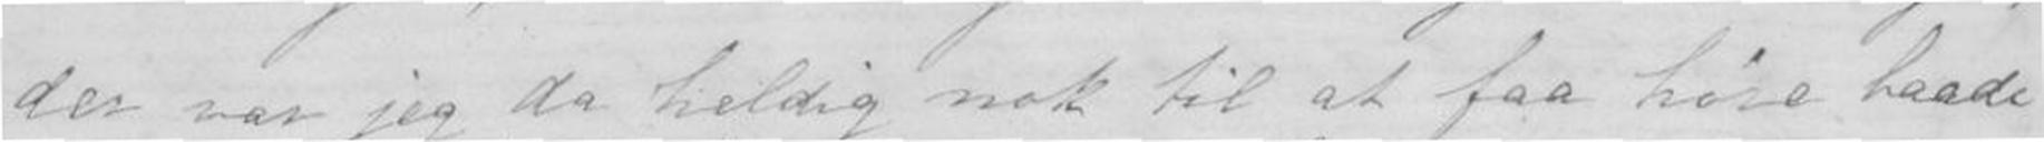der var jag da helsig nok at faa høre baade
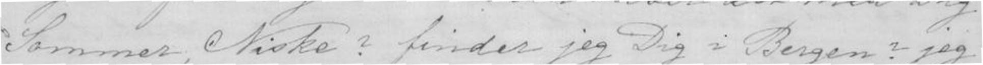Sommer, Niske? finder jeg Dig i Bergen? jeg
